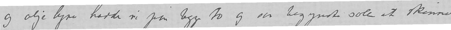og oljehyre havde vi paa begge to og saa begyndte solen at skinne
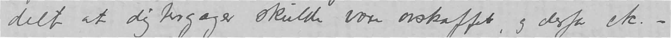delt at digtergager skulde være avskaffet, og derfor etc. –
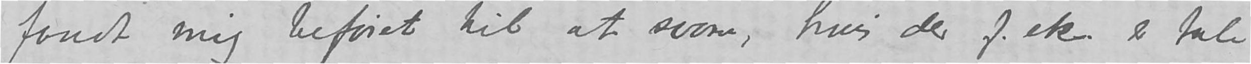fandt mig beføiet til atet svare, hvis der f.eks. er tale
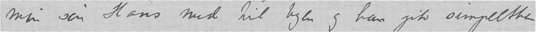min søn Hans med til byen og han gik simpelthen
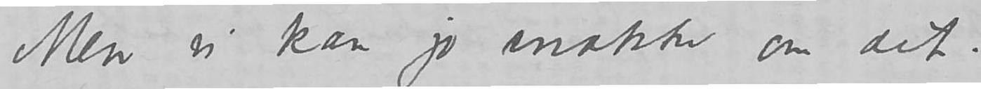Men vi kan jo snakke om det.
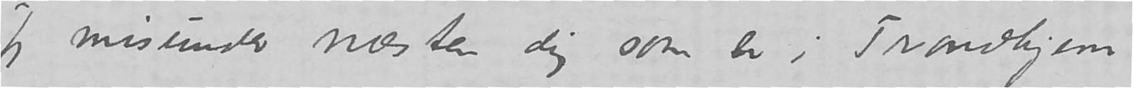Jeg misunder næsten dig som er i Trondhjem.
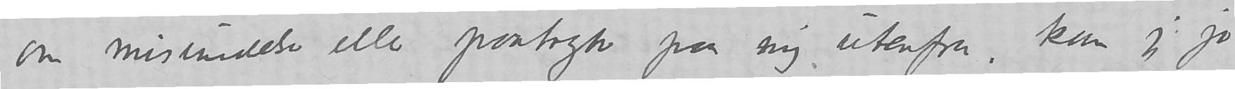om misundelse eller paatryk paa mig utenfra, kan jeg jo
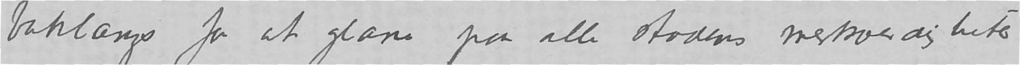baklængs for at glane paa alle stadens merkverdigheter
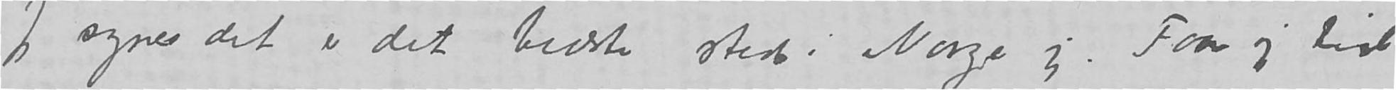Jeg synes det er det bedste sted i Norge jeg.  Faar jeg tid
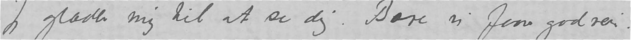Jeg glæder mig til at se dig.  Bare vi faar godveir.
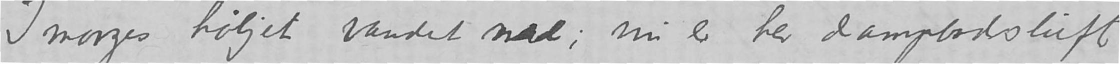Imorges høljet vandet ned; nu er her dampbadsluft og
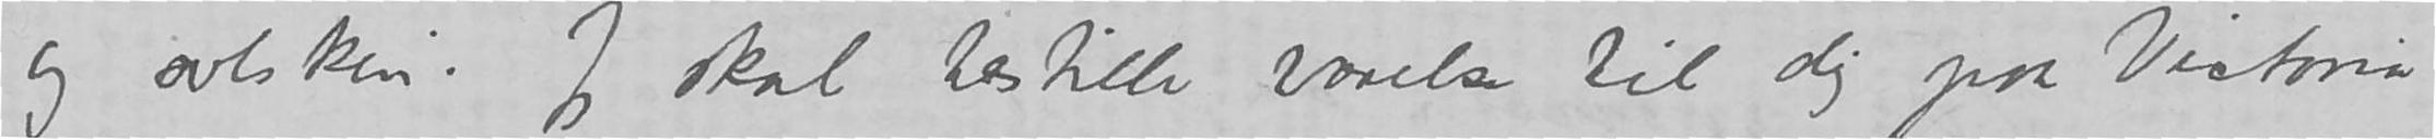solskin. Jeg skal bestille værelse til dig på Victoria
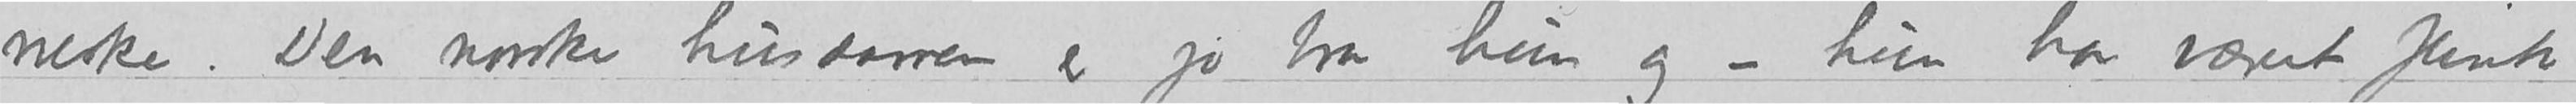neske. Den norske husdamen er jo bra hun og – hun har været flink
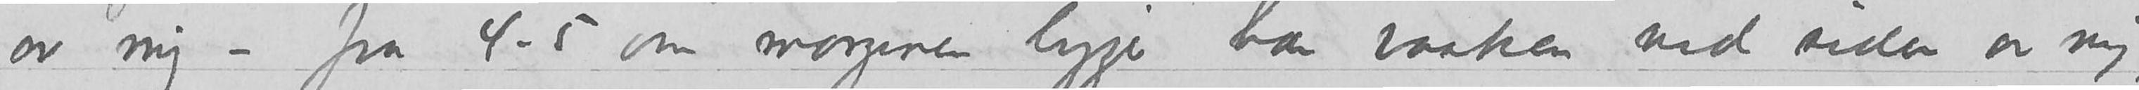av mig – fra 4-5 om morgenen ligger han vaaken ved siden av mig,
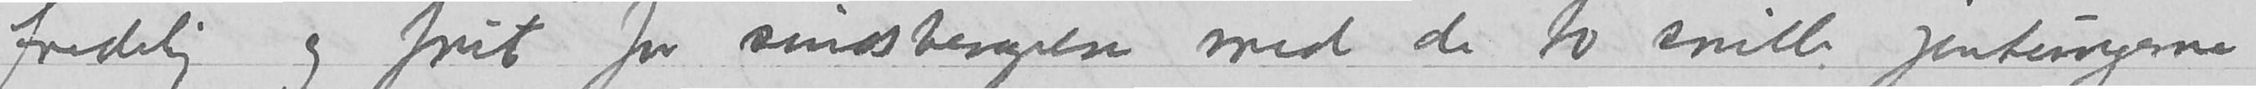fredelig og frit for sindsbevegelse med de to snille jentungerne
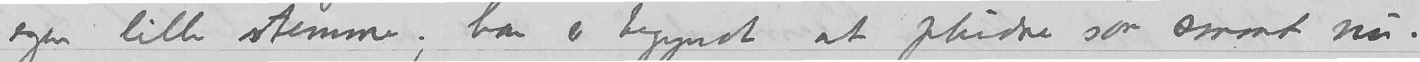egen lille stemme; han er begyndt at pludre saa smaat nu.
